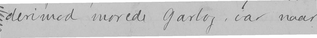derimod morede Garborg, var naar
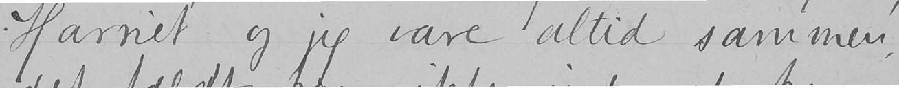Harriet og jeg vare altid sammen,
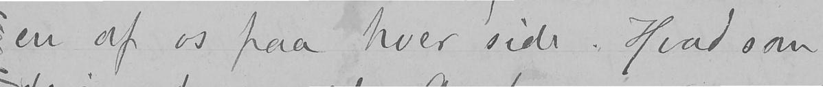en af os paa hver side. Hvad som
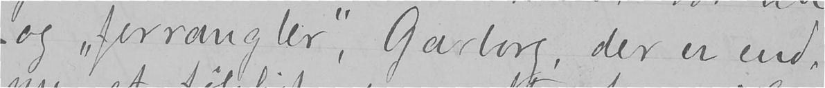og "forrangler", Garborg, der er end-
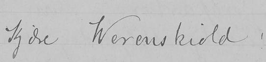Kjære Werenskiold!
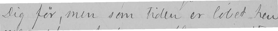Dig før, men som tiden er løbet hen
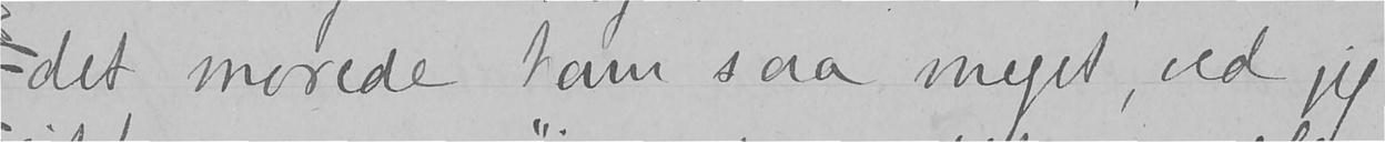det morede ham saa meget, ved jeg
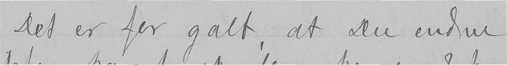Det er for galt, at Du endnu
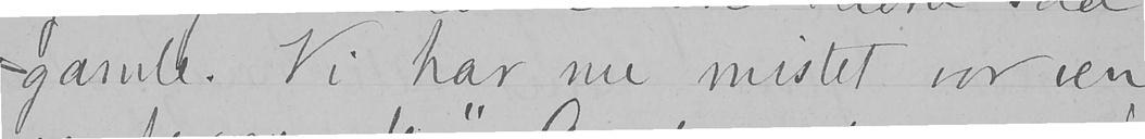gamle. Vi har nu mistet vor ven
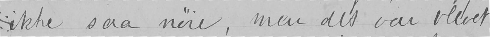ikke saa nøie, men det var blevet
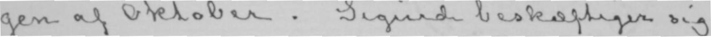gen af Oktober. Sigurd beskæftiger sig
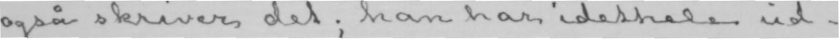også skriver det; han har idethele ud-
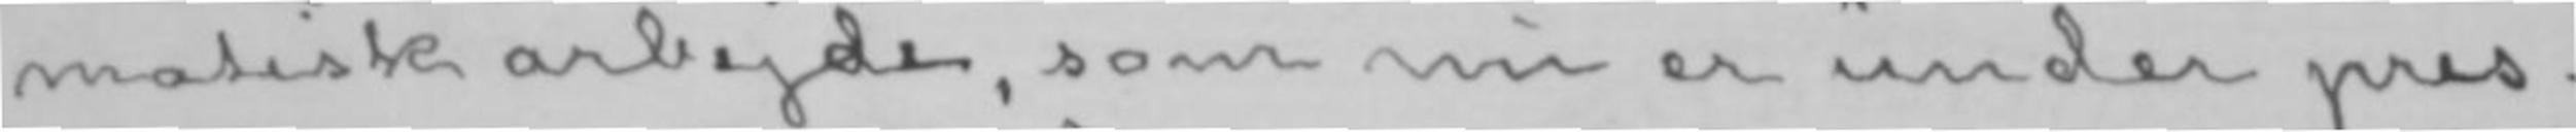matisk arbejde, som nu er under pres-
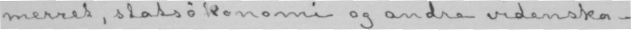merret, statsøkonomi og andre videnska-
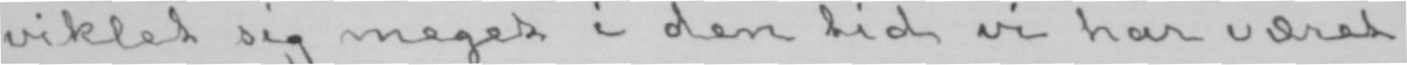viklet sig meget i den tid vi har været
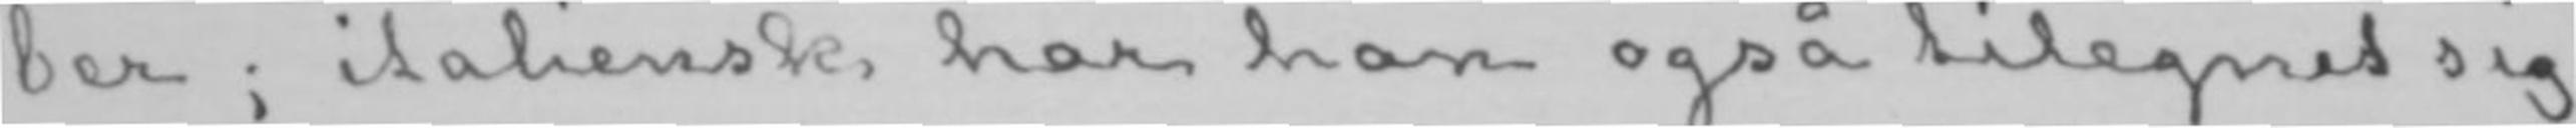ber; italiensk har han også tilegnet sig
